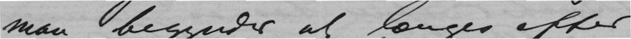man begynder at længes efter
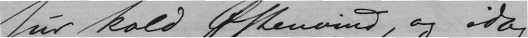sur kold Østenvind, og idag
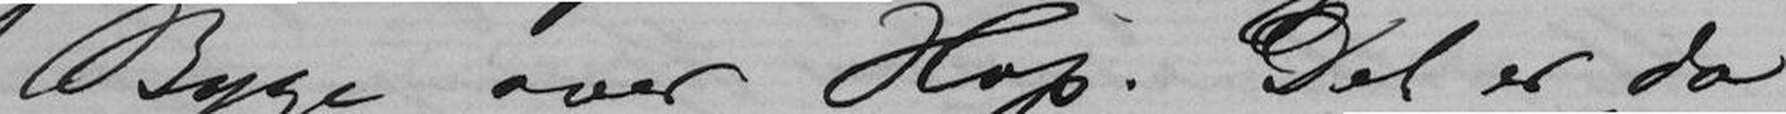Byge over Hop. Det er da
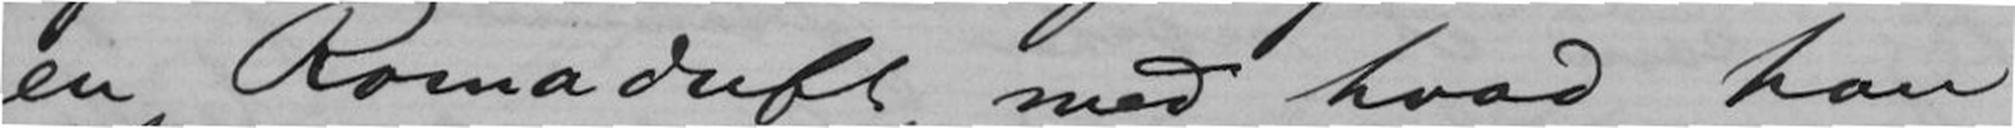en Romaduft med hvad han
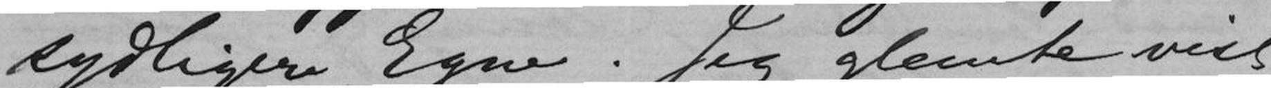sydligere Egne. Jeg glemte vist
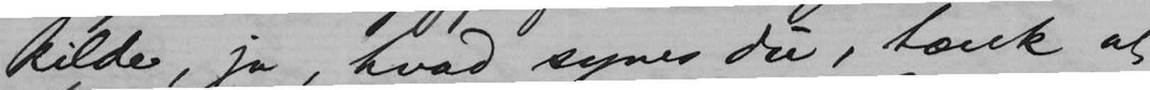kilde, ja, hvad synes du, tænk at
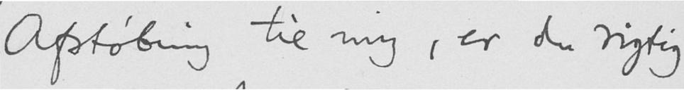Afstøbning til mig, er du rigtig
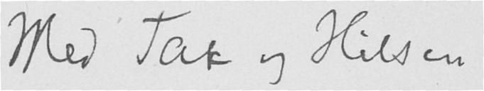Med Tak og Hilsen
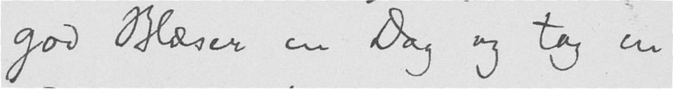god Blæser en Dag og tag en
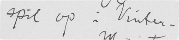spil op i Vinter.
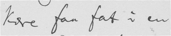Kære faa fat i en
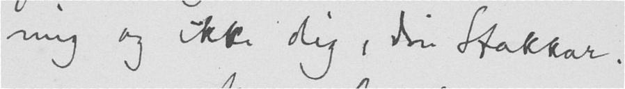mig og ikke dig, din Stakkar.
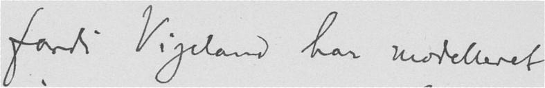fordi Vigeland har modelleret
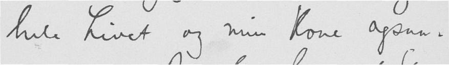hele Livet og min Kone ogsaa.
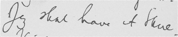Jeg skal have et Skue-
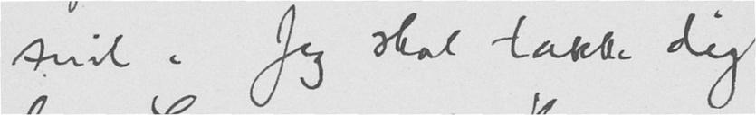snil. Jeg skal takke dig
